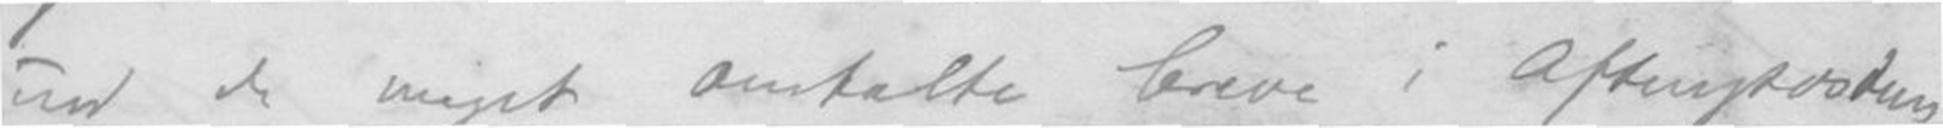ud de meget omtalte breve i Aftenpostens
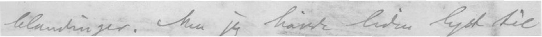blandinger. Men jeg havde liden lyst til
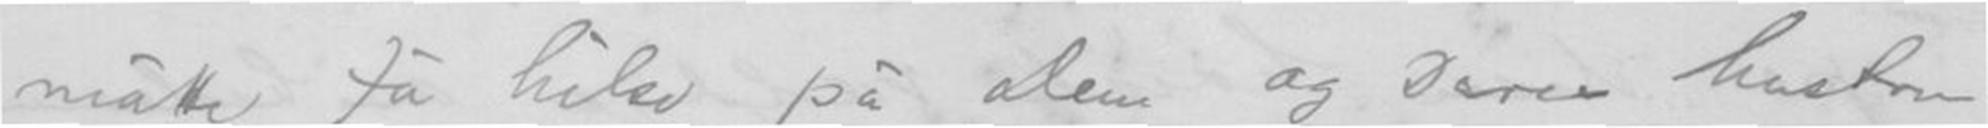måtte få hilse på Dem og Deres hustru
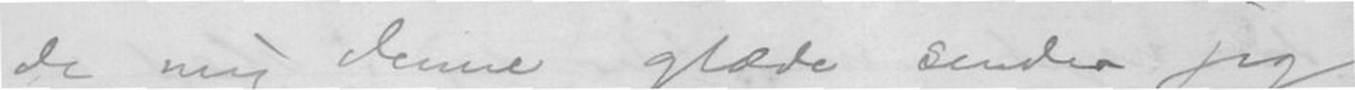de mig denne glæde sender jeg
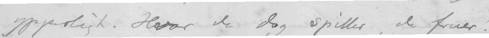ypperligt. Hvor de dog spiller, de fruer!
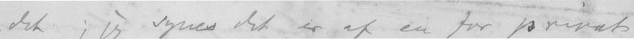det; jeg synes det er af en for privat
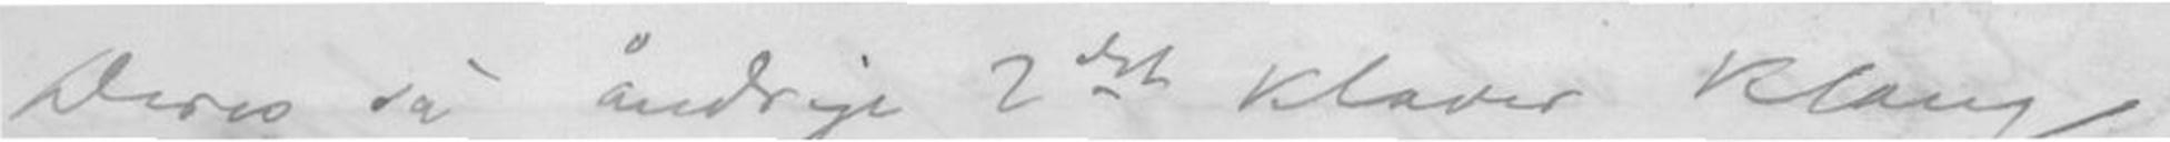Deres så åndrige 2det Klaver klang
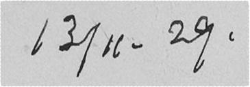13/11. 29.
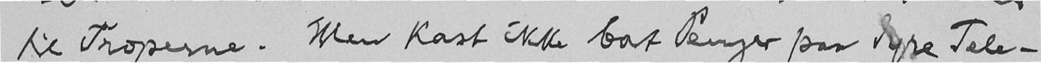til Troperne. Men kast ikke bort Penger paa dyre Tele-
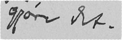gjøre det.
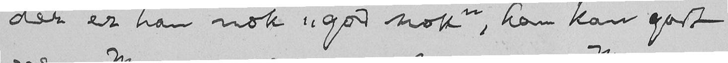der er han nok "god nok", han kan godt
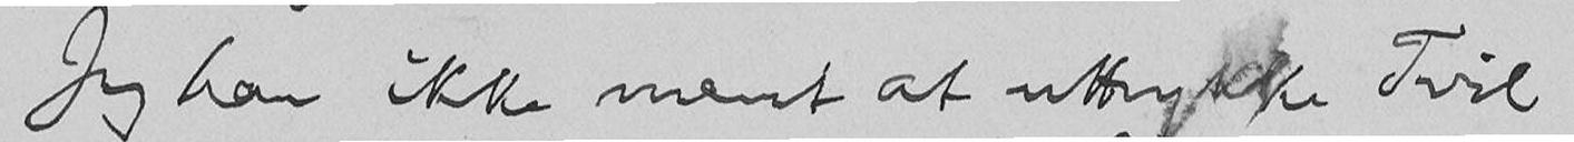Jeg har ikke ment at uttrykke Tvil
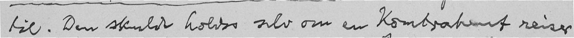til. Den skulde holdes selv om en Kontrahent reiser
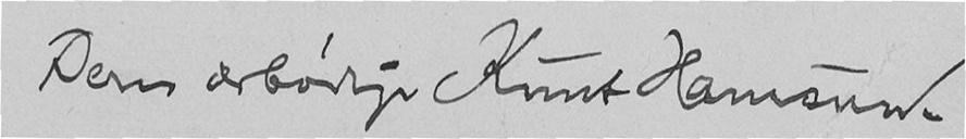Deres ærbødige Knut Hamsun.
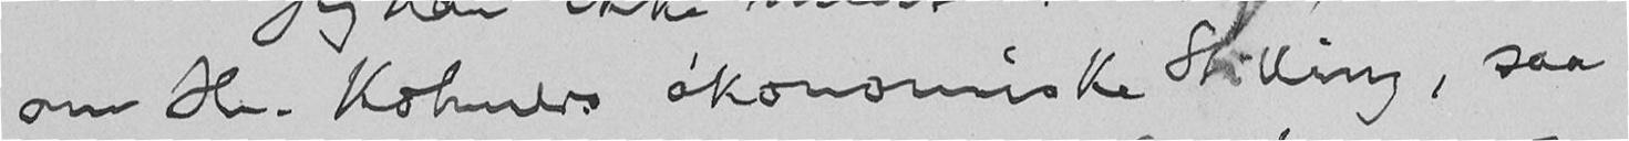om Hr. Kohners økonomiske Stilling, saa
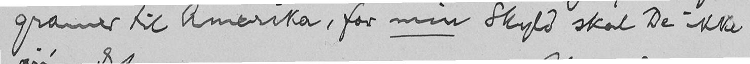gramer til Amerika, for min Skyld skal De ikke
